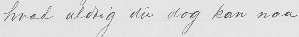hvad aldrig du dog kan naa
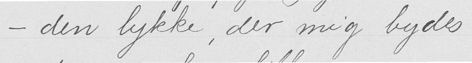- den lykke der mig bydes
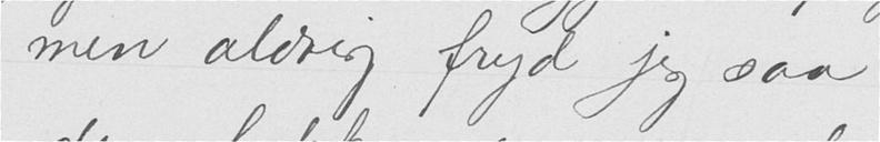men aldrig fryd jeg saa
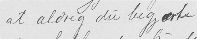at aldrig du begjærte
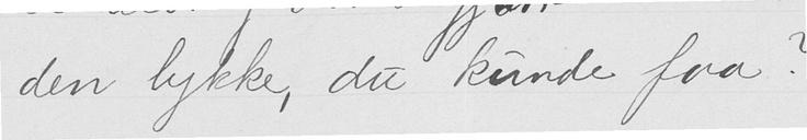den lykke, du kunde faa?
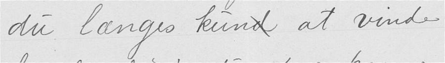du længes kund at vinde
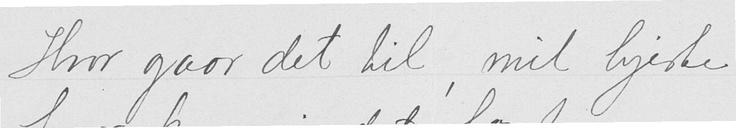Hvor gaar det til, mit hjerte,
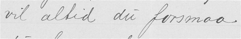vil altid du forsmaa,
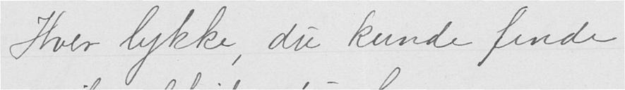Hver lykke, du kunde finde
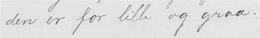den er for lille og graa.
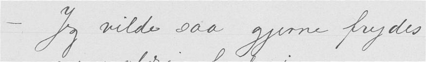- Jeg vilde saa gjerne frydes
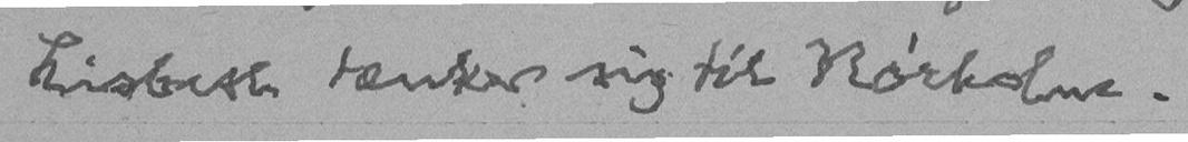Lisbeth tænker sig til Nørholm.
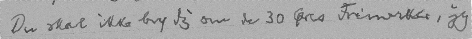Du skal ikke bry dig om de 30 Øres Frimerker, jeg
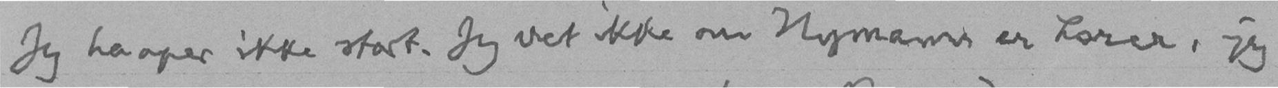Jeg haaper ikke stort. Jeg vet ikke om Nymann er Lærer, jeg
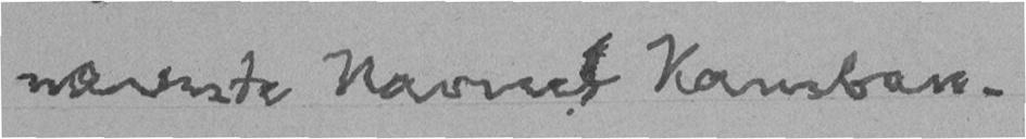nævnte Navnet Kamban.
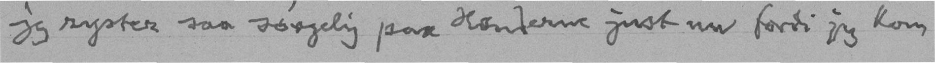jeg ryster saa sørgelig paa Hænderne just nu fordi jeg kom
